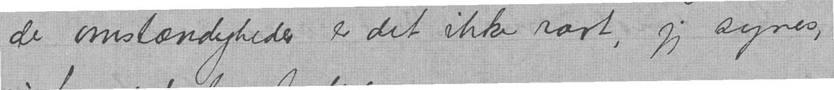de omstændigheder er det ikke rart, jeg synes,
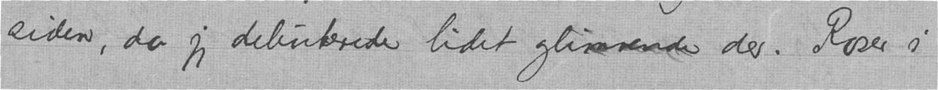siden, da jeg debuterede lidet glimrende der. Roser i
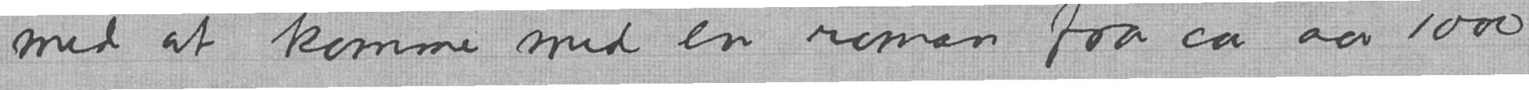med at komme med en roman fra ca. aar 1000
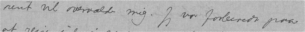rent vil overvælde mig. Jeg var forberedt paa
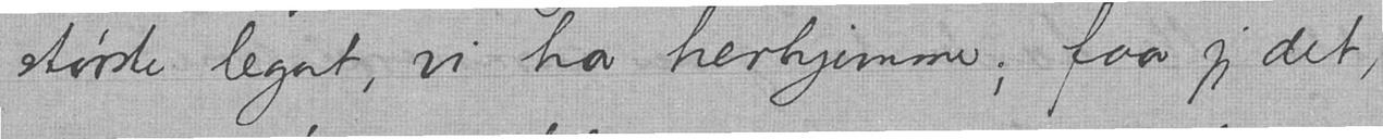største legat, vi har herhjemme; faar jeg det,
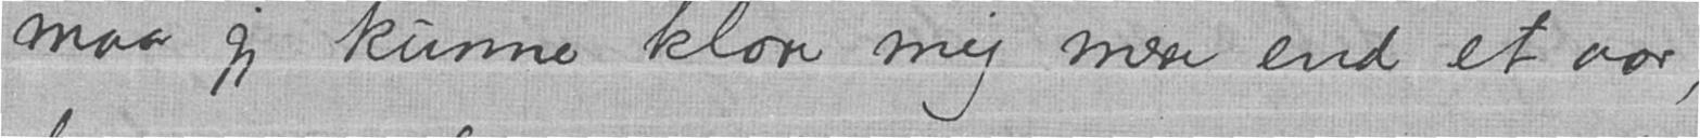maa jeg kunne klare mig mere end et aar,
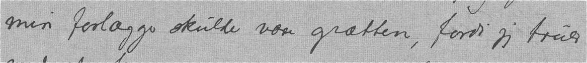min forlægger skulde være grætten, fordi jeg truer
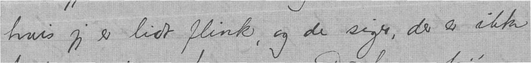hvis jeg er lidt flink, og de siger, der er ikke
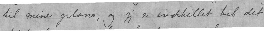til mine planer, og jeg er indstillet til det
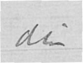din
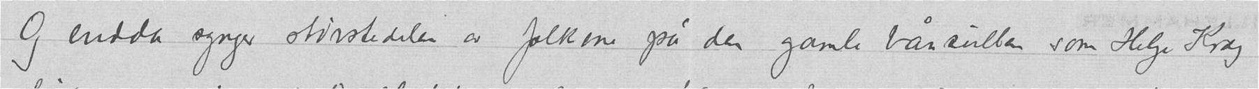Og endda synger størstedelen av folkene på den gamle bånsullen som Helge Krog
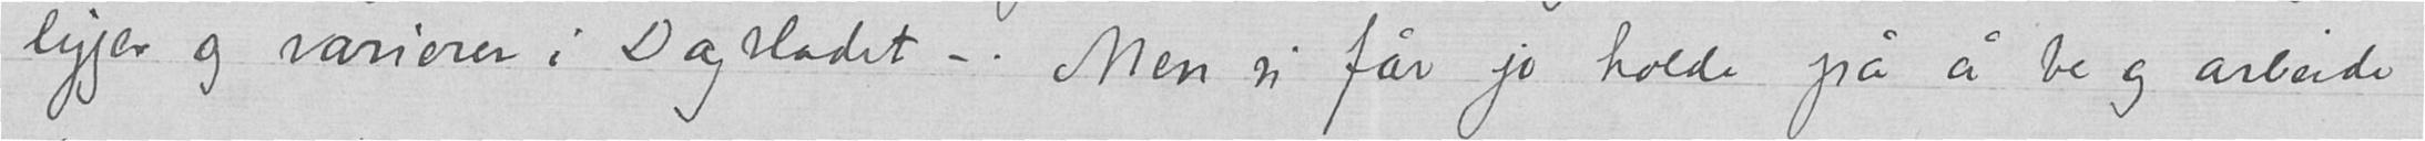ligger og varierer i Dagbladet –. Men vi får jo holde på å be og arbeide
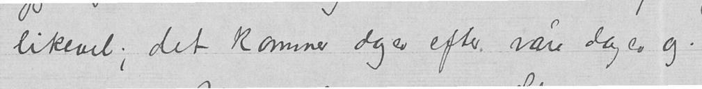likevel; det kommer dager efter våre dager og.
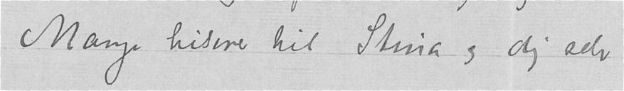Mange hilsener til Stina og dig selv
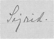Sigrid.
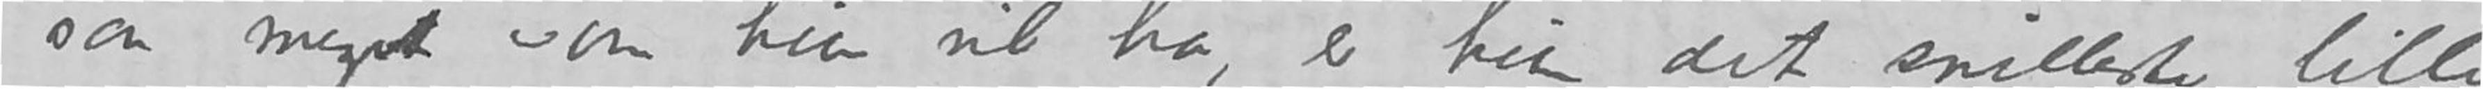saa meget som hun vil ha, er hun det snilleste lille
